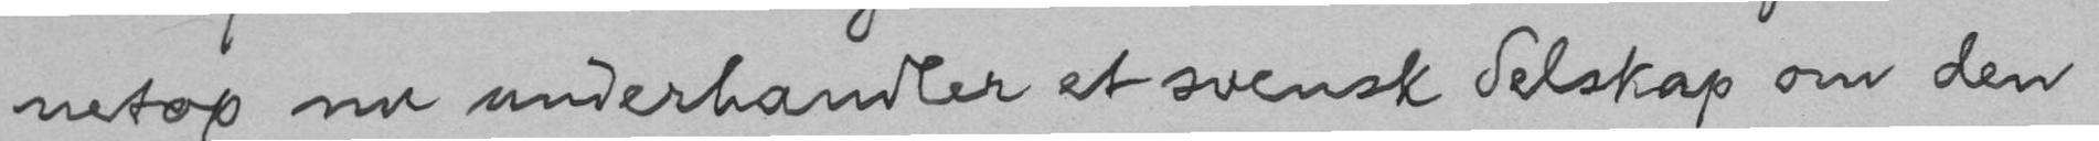netop nu underhandler et svensk Selskap om den
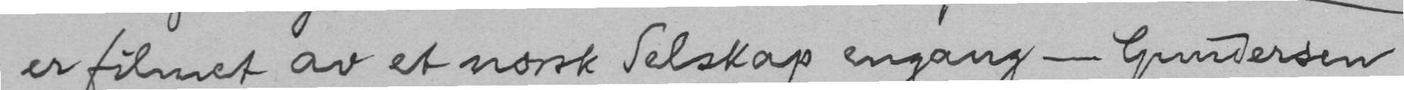er filmet av et norsk Selskap engang - Gundersen
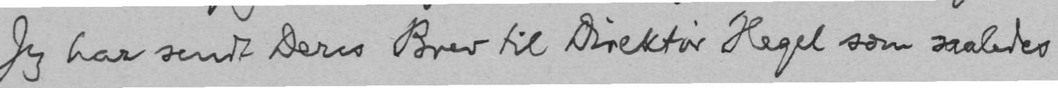Jeg har sendt Deres Brev til Direktør Hegel som saaledes
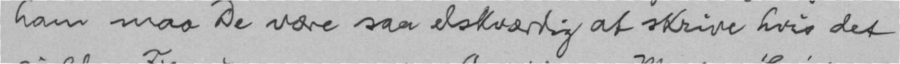ham maa De være saa elskværdig at skrive hvis det
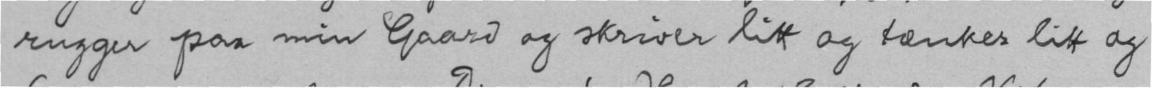rugger paa min Gaard og skriver litt og tænker litt og
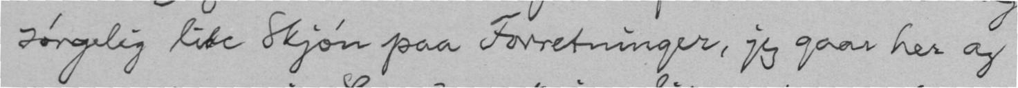sørgelig lite Skjøn paa Forretninger, jeg gaar her og
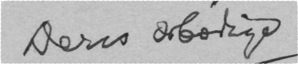Deres ærbødige
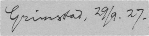Grimstad, 29/9. 27.
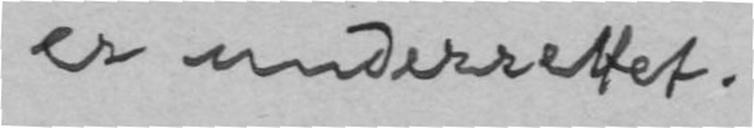er underrettet.
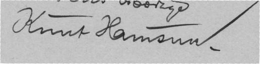Knut Hamsun.
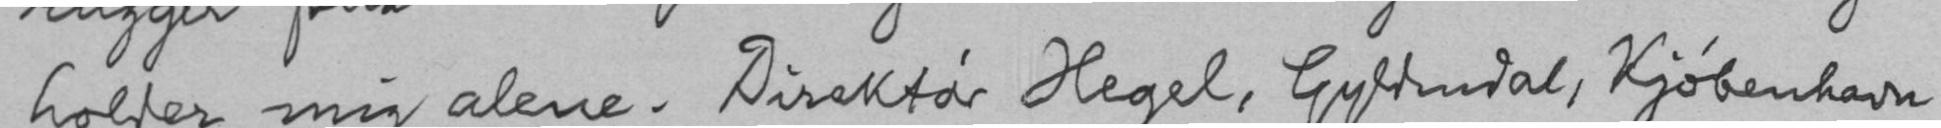holder mig alene. Direktør Hegel, Gyldendal, Kjøbenhavn
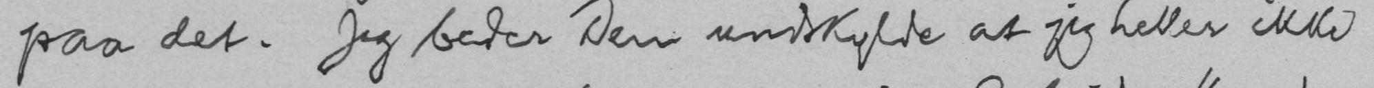paa det. Jeg beder Dem undskylde at jeg heller ikke
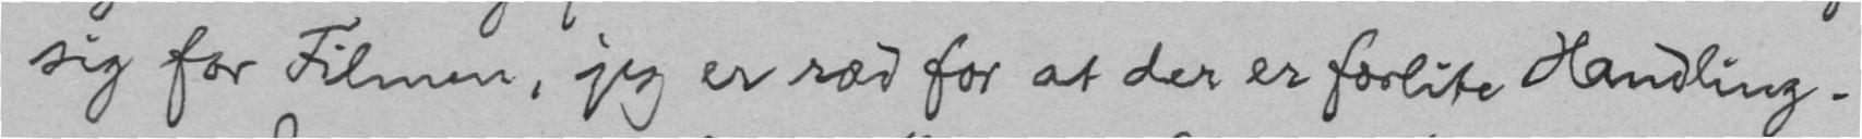sig for Filmen, jeg er ræd for at der er forlite Handling.
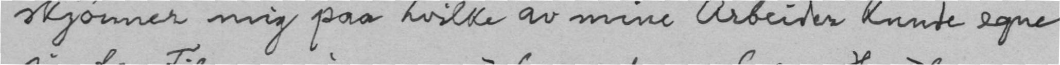skjønner mig paa hvilke av mine Arbeider kunde egne
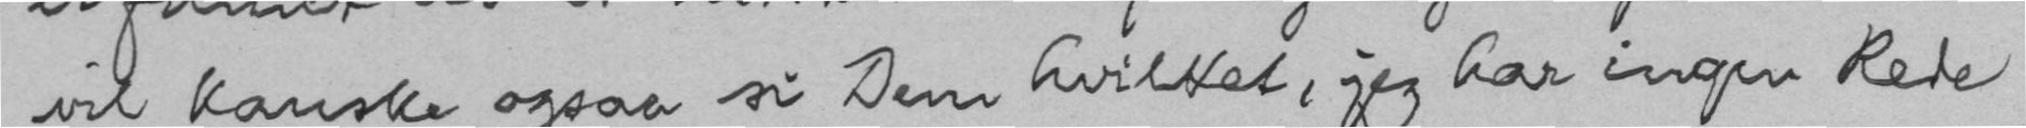vil kanske ogsaa si Dem hvilket, jeg har ingen Rede
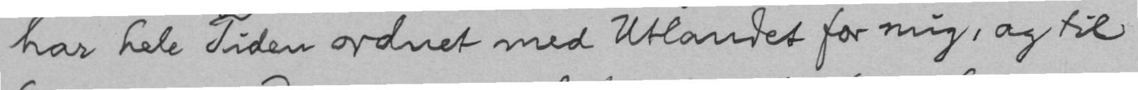har hele Tiden ordnet med Utlandet for mig, og til
